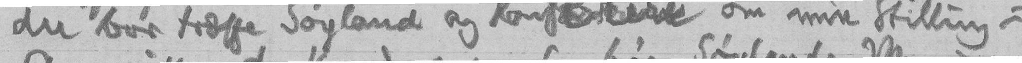du bør træffe Søyland og konferere om min Stilling i
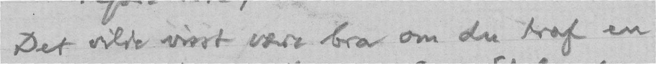Det vilde visst være bra om du traf en
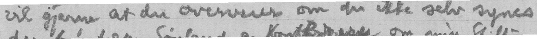vil gjerne at du overveier om du ikke selv synes
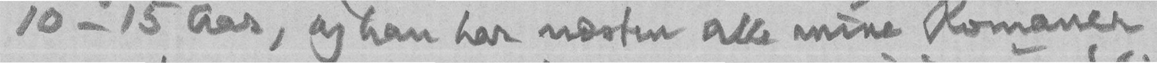10-15 Aar, og han har næsten alle mine Romaner
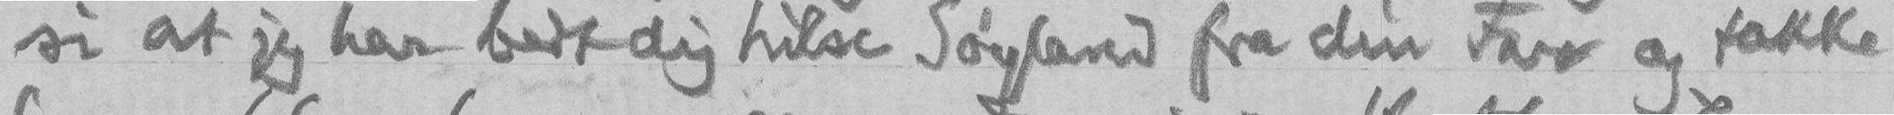si at jeg har bedt dig hilse Søyland fra din Far og takke
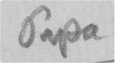Papa
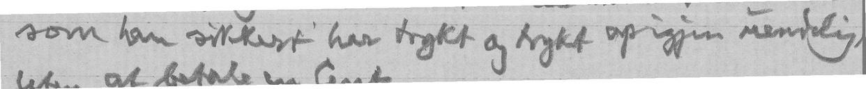som han sikkert har trykt og trykt op igjen uendelig,
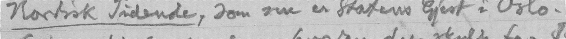Nordisk Tidende, som nu er Statens Gjest i Oslo.
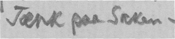Tænk paa Saken.
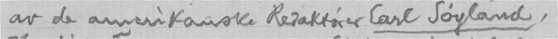av de amerikanske Redaktører Carl Søyland,
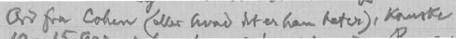Ord fra Cohen (eller hvad det er han heter), kanske
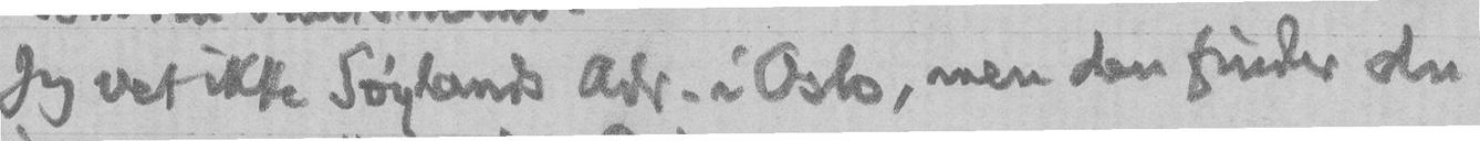Jeg vet ikke Søylands Adr. i Oslo, men den finder du
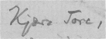Kjære Tore,
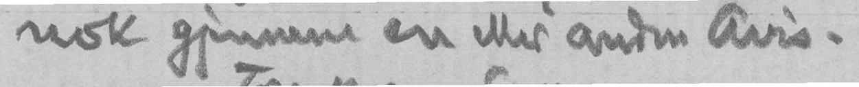nok gjennem en eller anden Avis.
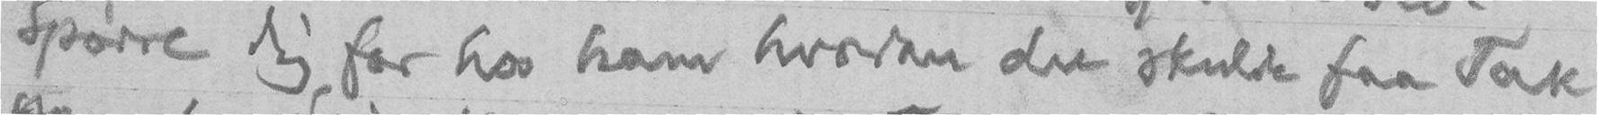Spørre dig for hos ham hvordan du skulde faa Tak
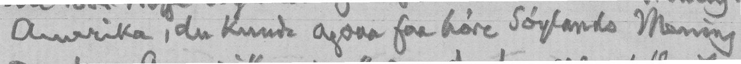Amerika, du kunde ogsaa faa høre Søylands Mening
